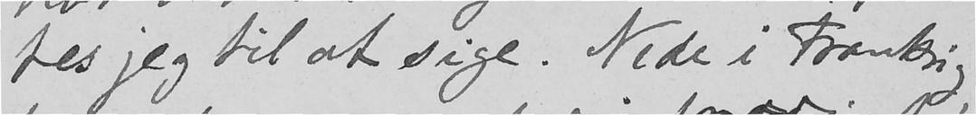tes jeg til at sige. Nede i Frankrig,
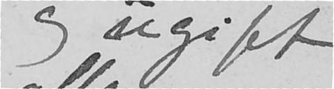og ugift
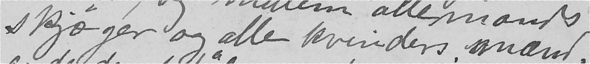skjøger og alle kvinders mænd
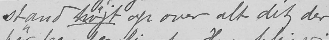stand højt op over alt det, der
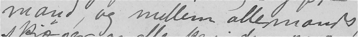mand, og mellem allemands
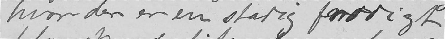hvor der er en stadig frodigt
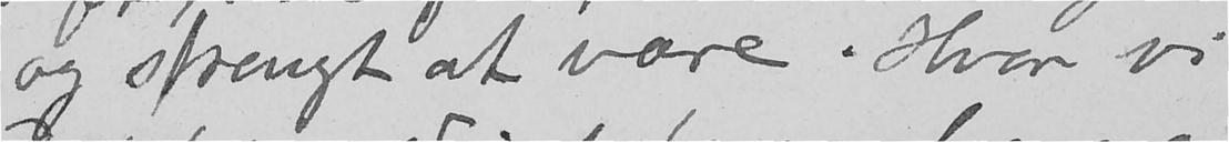og strengt at være. Hvor vi
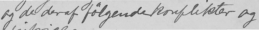og de deraf følgende konflikter og
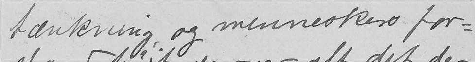tænkning, og menneskers for-
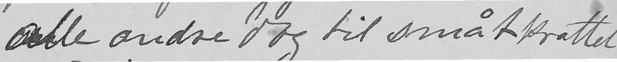alle andre dog til småtkrattet
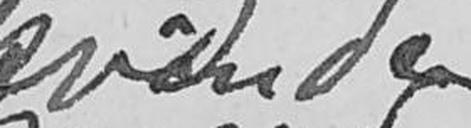kvinde
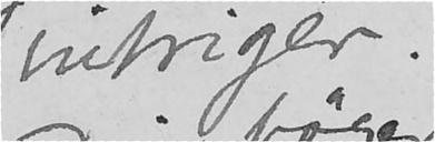intriger.
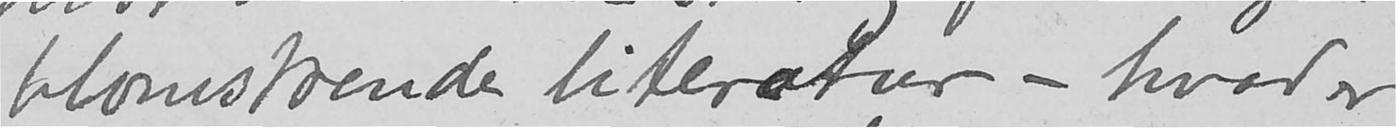blomstrende literatur - hvad er
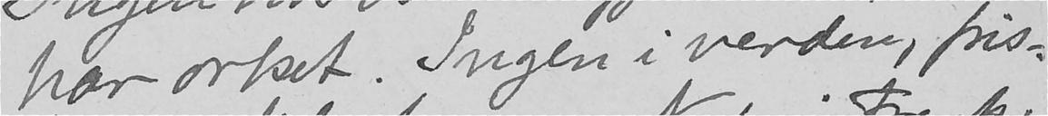har orket. Ingen i verden, fris-
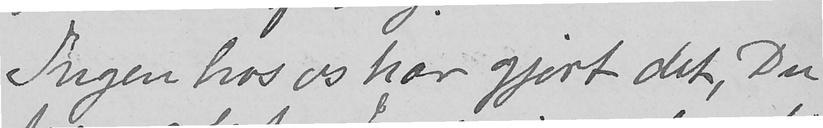Ingen hos os har gjort det, Du
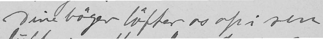Dine bøger løfter os op i ren
